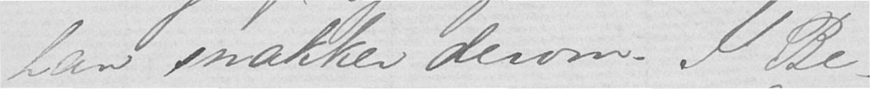han snakker derom. I Be-
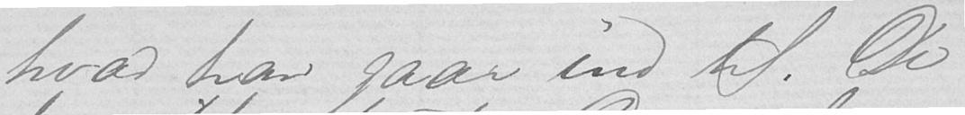hvad han gaar ind til. Du
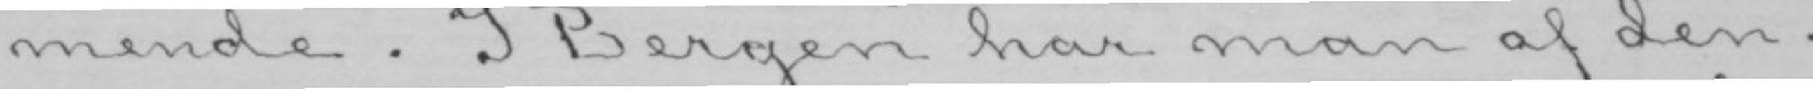mende. I Bergen har man af den-
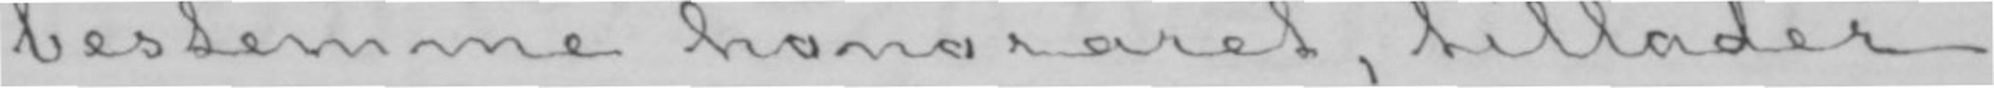bestemme honoraret, tillader
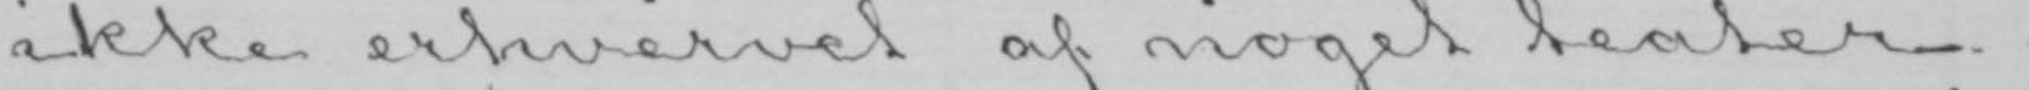ikke erhvervet af noget teater.
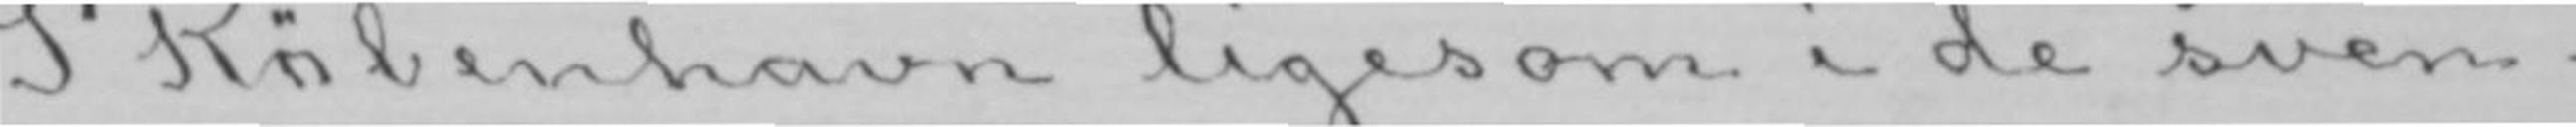I København ligesom i de sven-
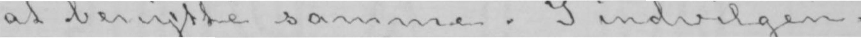at benytte samme. I indvilgen-
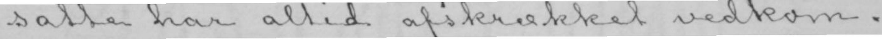satte har altid afskrækket vedkom-
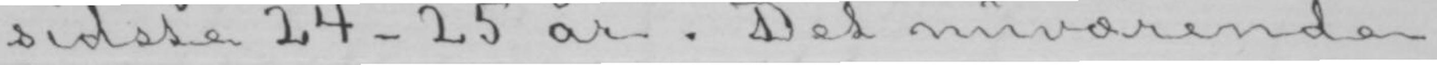sidste 24-25 år. Det nuværende
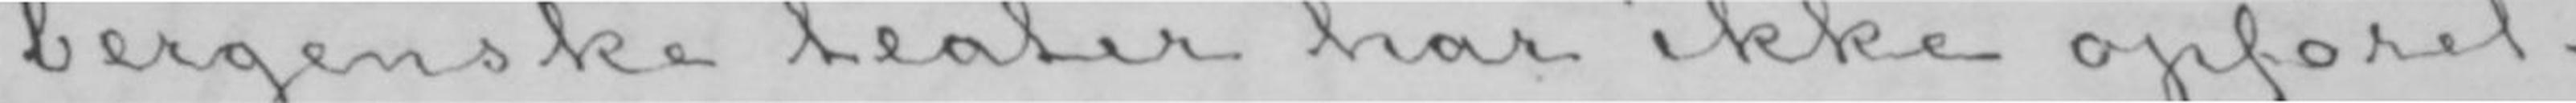bergenske teater har ikke opførel-
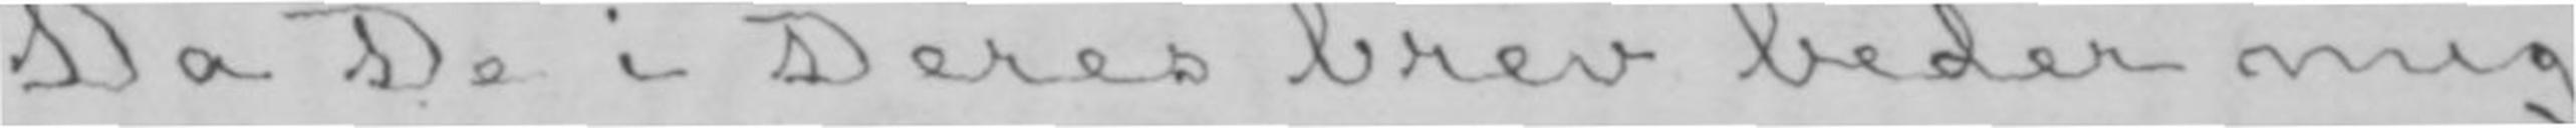Da De i Deres brev beder mig
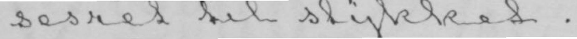sesret til stykket.
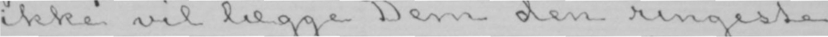ikke vil lægge Dem den ringeste
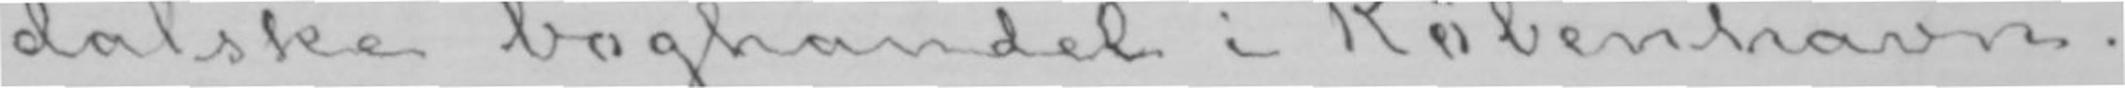dalske boghandel i København.
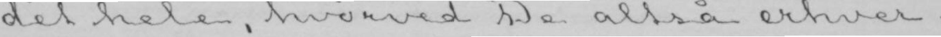det hele, hvorved De altså erhver-
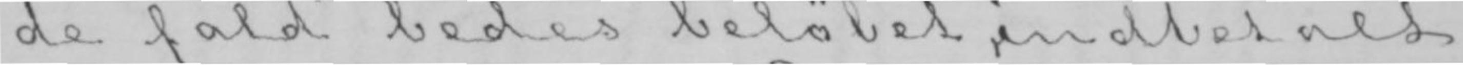de fald bedes beløbet indbetalt
